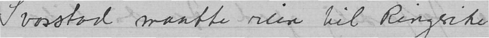Svarstad maatte reise til Ringerike
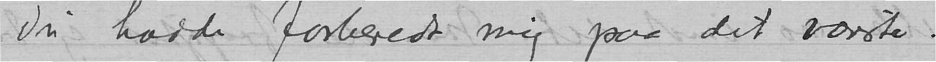Du hadde forberedt mig paa det værste.
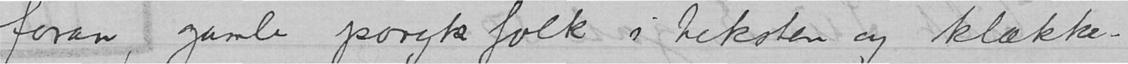foran, gamle parykfolk i teksten og klække-
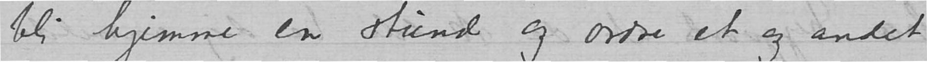bli hjemme en stund og ordne et og andet
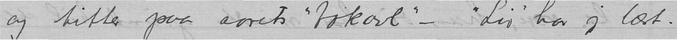og titter paa aarets < «bokavl» > -  <«Liv»>har jeg læst.
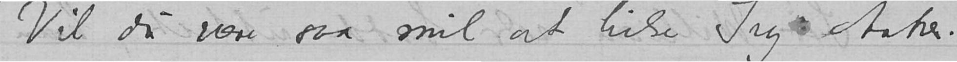Vil du være saa snil at hilse Ing. Anker.
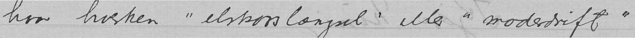hvar hverken «elskovslængsel» eller «moderdrift»
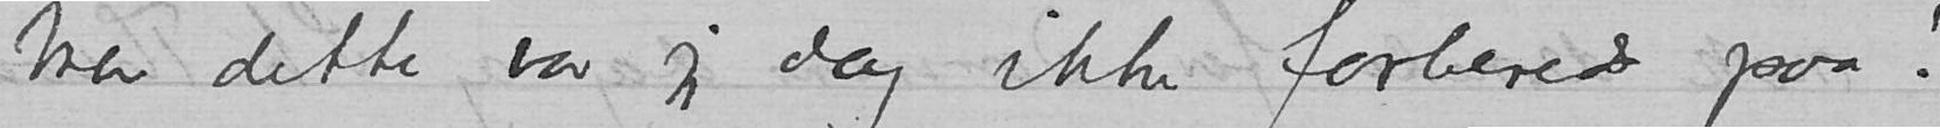Men dette var jeg  dog ikke forberedt paa!
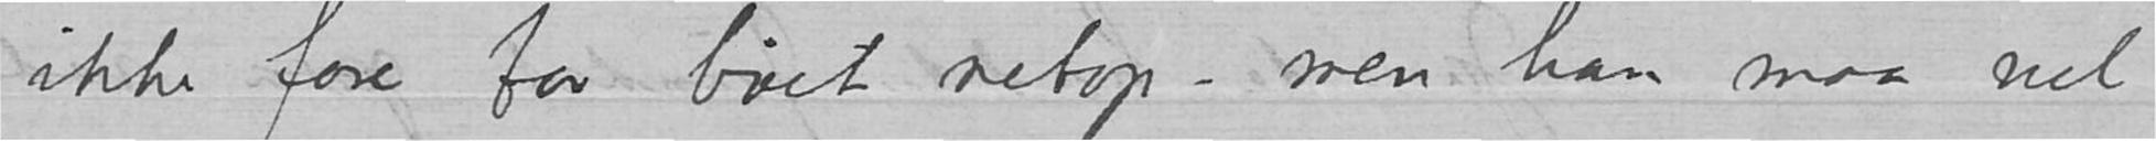ikke fare for livet netop – men han maa vel
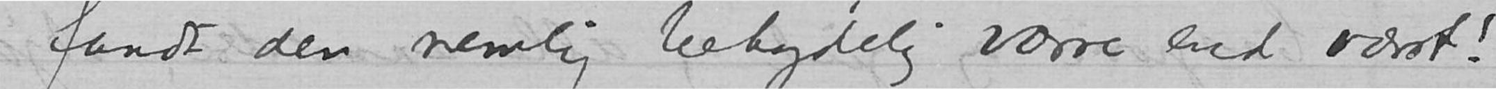Jeg fandt den nemlig betydelig værre end værst!
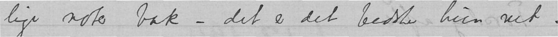lige noter bak – det er det bedste hun vet.
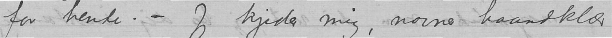for hende. – Jeg kjeder mig, navner haandklær
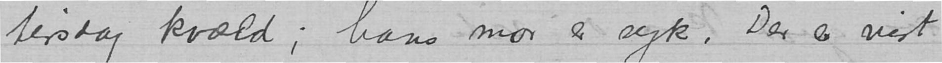tirsdag kvæld ; hans mor er syk. Der er vist
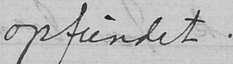opfundet.
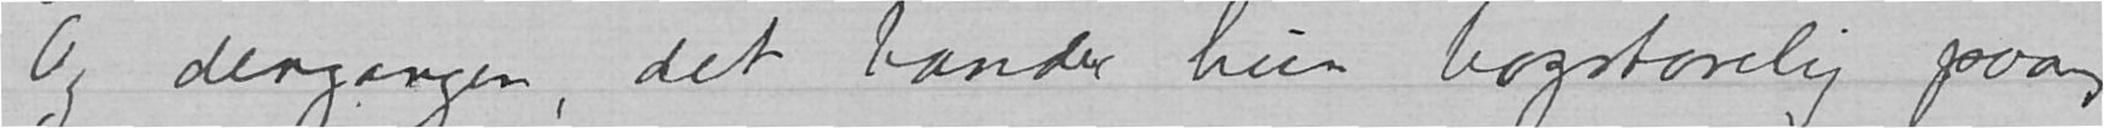Og dengangen, det bander hun bogstavelig paa<,>
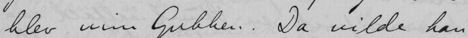blev min Gubbe. Da vilde han
